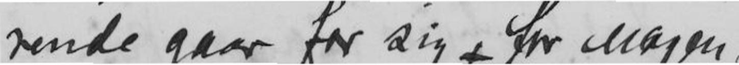rende gaar for sig - for nogen,
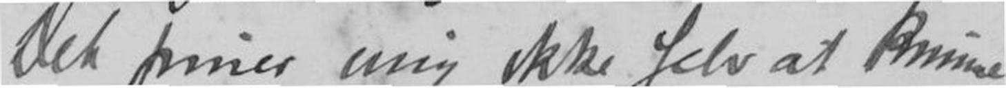Det piner mig ikke selv at kunne
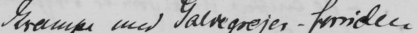krampe med Galdegrejer -foruden
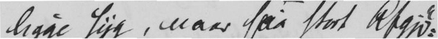ligge syg, naar saa stort Afgjø-
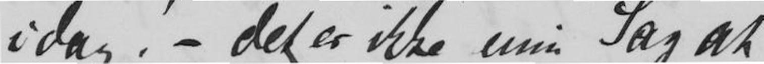idag! - det er ikke min Sag at
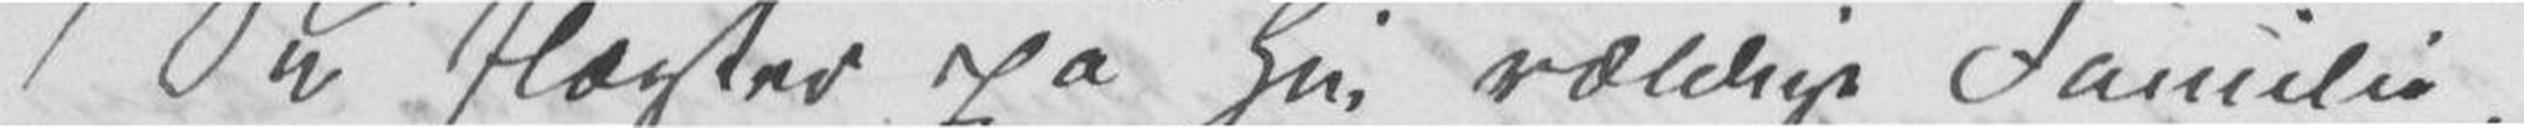Du slægter på Hin vældige Familie,
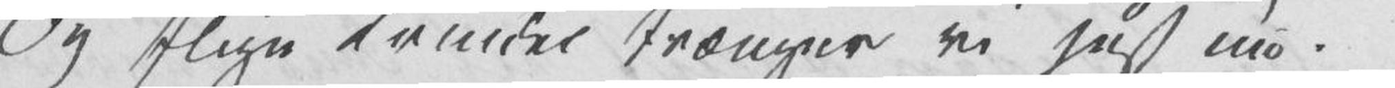Og slige Kvinder trænger vi just nu.
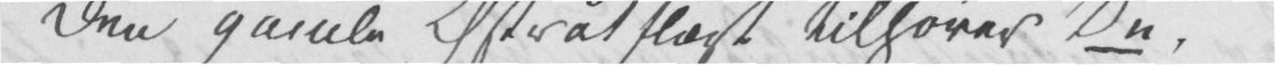Den gamle Østråtslægt tilhører Du,
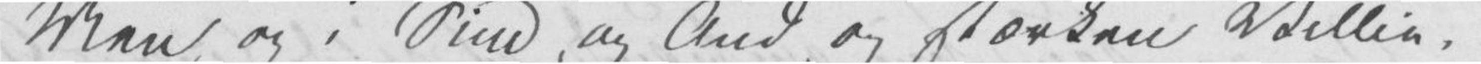Men og i Sind og Ånd og stærken Villie,
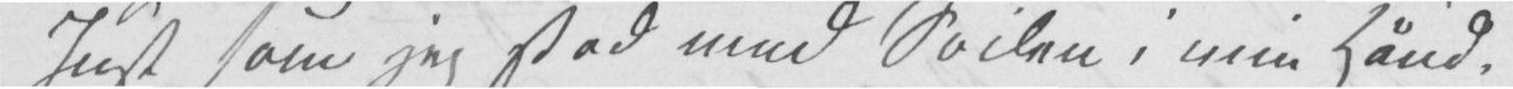Just som jeg stod med Søilen i min Hånd.
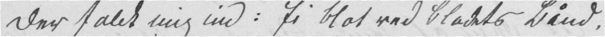Der faldt mig ind: Ei blot ved Blodets Bånd,
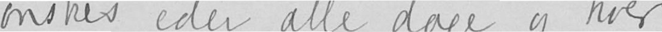ønskes eder alle dage og hver
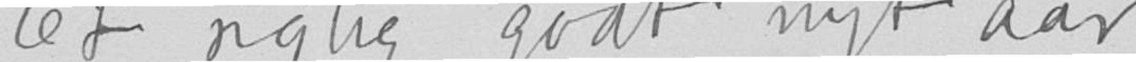Et rigtig godt nyt aar
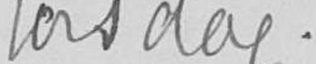torsdag.
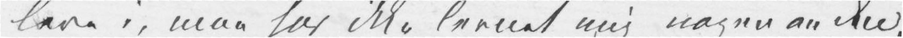leve i, man har ikke levnet mig nogen anden,
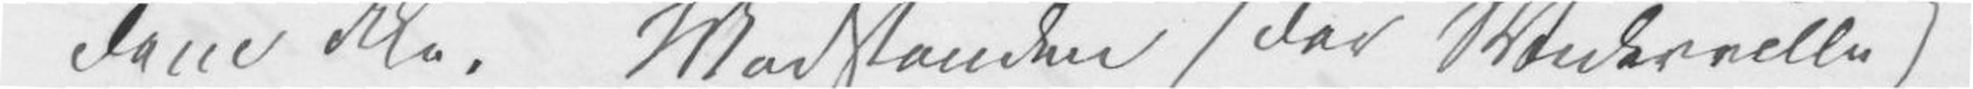dem ikke. Modstanden (der Widerwille)
